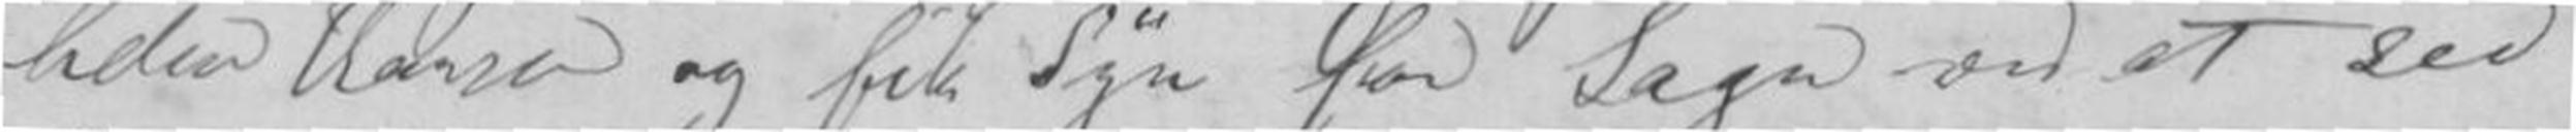helm Hansen og fik Syn for Sagen om at see
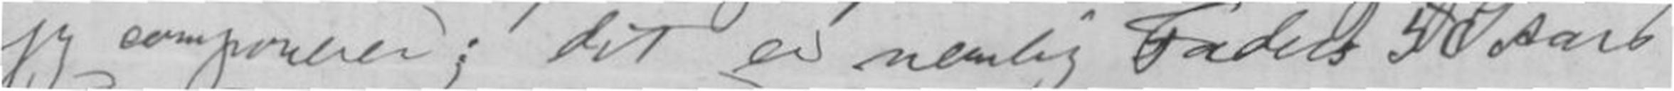jeg componerer; det er nemlig Faders 50 Aars
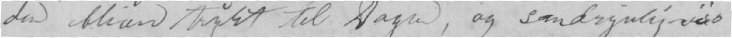den bliver trykt til Dagen, og sandsynligvis
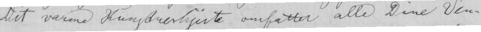Dit varme Kunstnerhjerte omfatter alle Dine Ven-
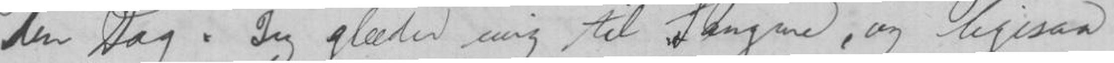den Dag. Jeg glæder mig til Sangene, og ligesaa
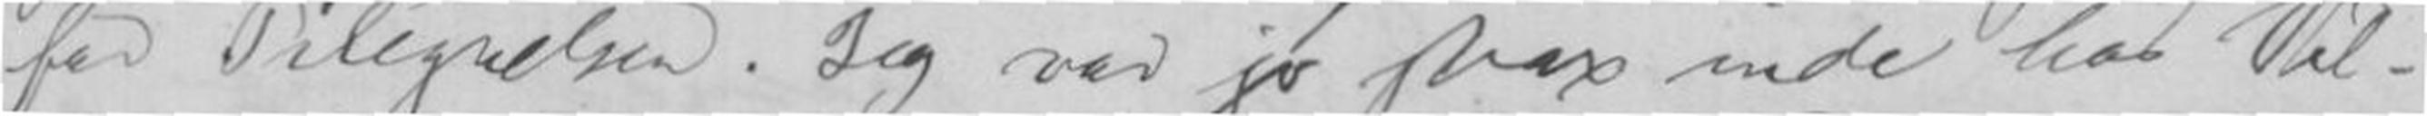for Tilegnelsen. Jeg var jo strax inde hos Vil-
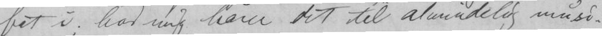fat i; hos mig hører det til almindelig musi-
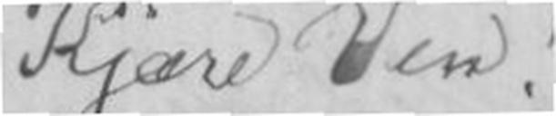Kjære Ven!
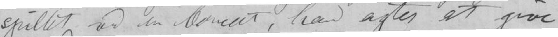spillet ved en Concert, han agter at give
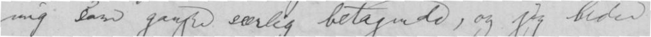mig som ganske særlig betagende, og jeg beder
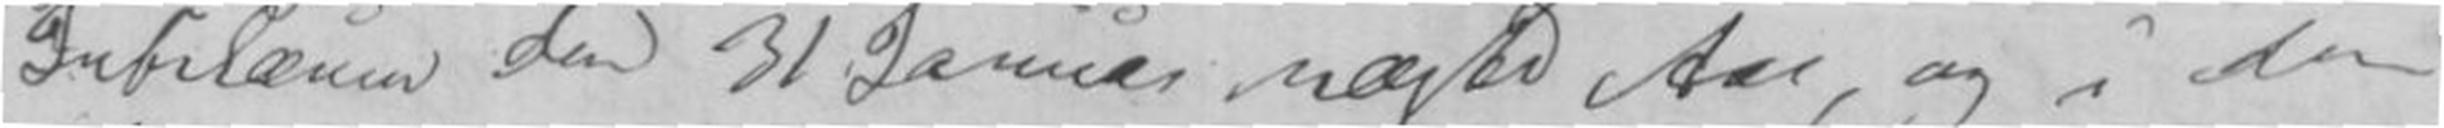Jubilæum den 31 Januar næste Aar, og i den
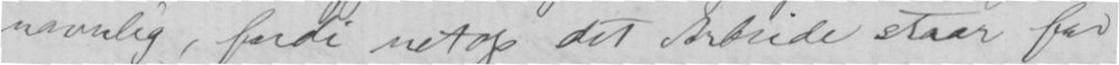navnlig, fordi netop det Arbeide staar for
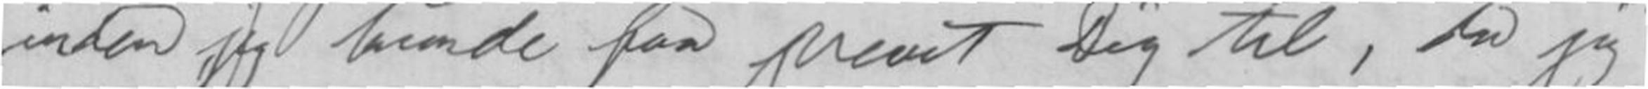inden jeg kunde faa skrevet Dig til, da jeg
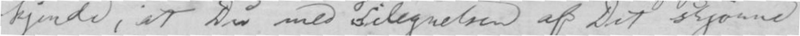kjende, at Du med Tilegnelsen af Dit skjønne
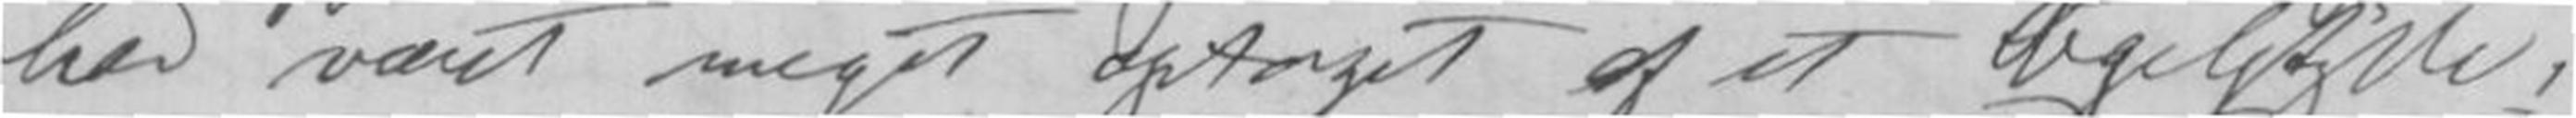har været meget optaget af et Orgelstykke,
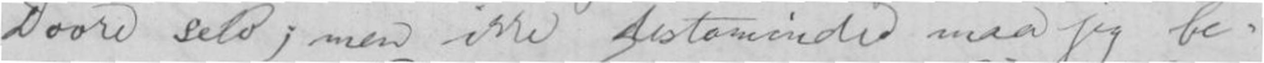Dovre selv; men ikke destomindre maa jeg be-
